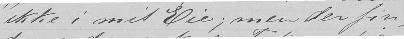ikke i mit Eie; men der fin-
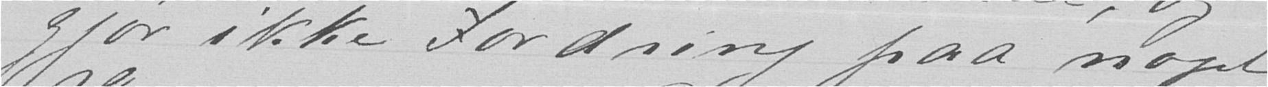gjør ikke Fordring paa noget
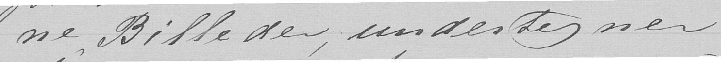ne Billeder, undertegner
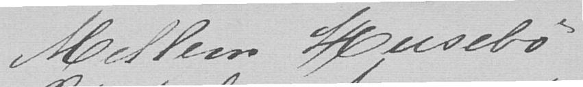Mellem Husebø
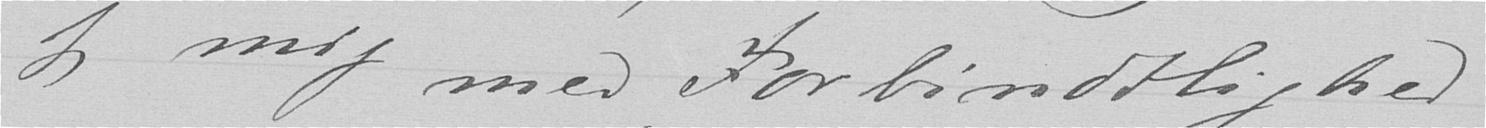jeg mig med Forbindtlighed
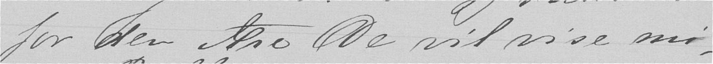for den Ære De vil vise mi-
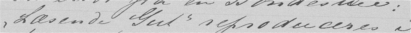"Læsende Gut" reproduceres i
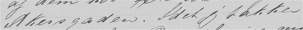Akersgaden. Idet jeg takker
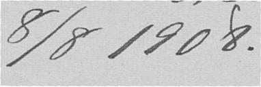8/8 1908.
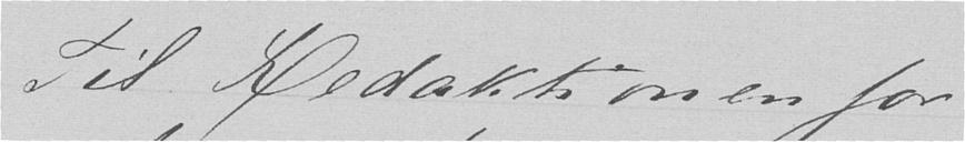Til Redaktionen for
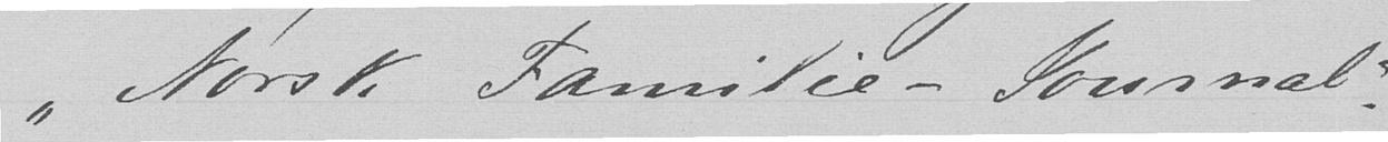"Norsk Familie-Journal"
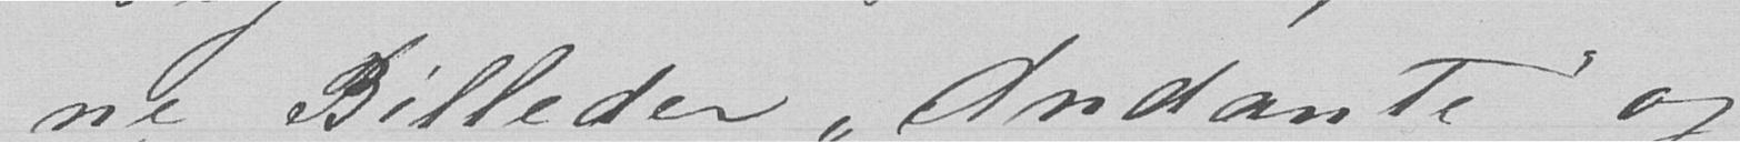ne Billeder "Andante" og
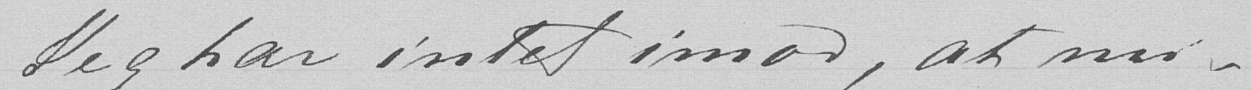Jeg har intet imod, at mi-
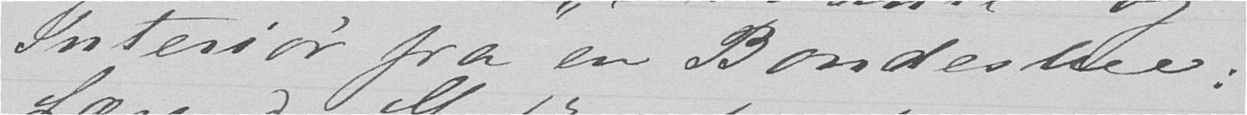Interiør fra en Bondestue:
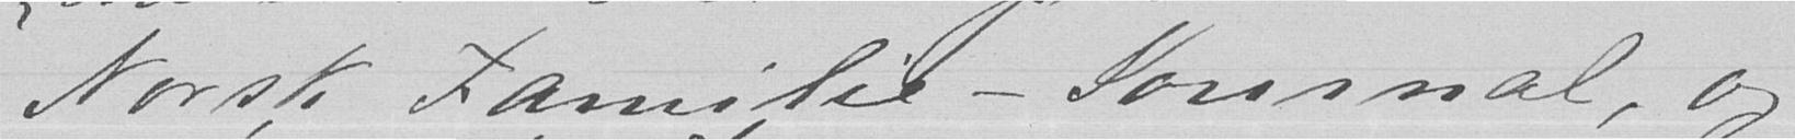Norsk Famile-Jorirnal, og
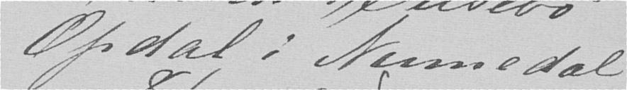Opdal i Numedal
